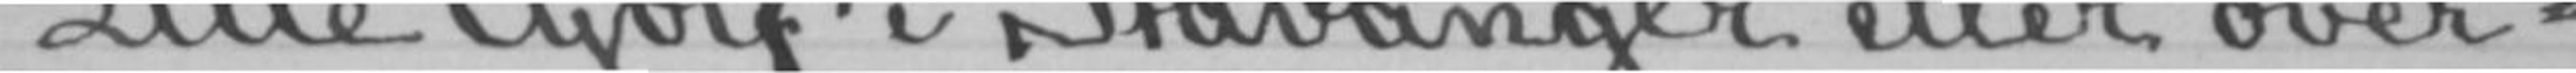«Lille Eyolf» i Stavanger eller over-
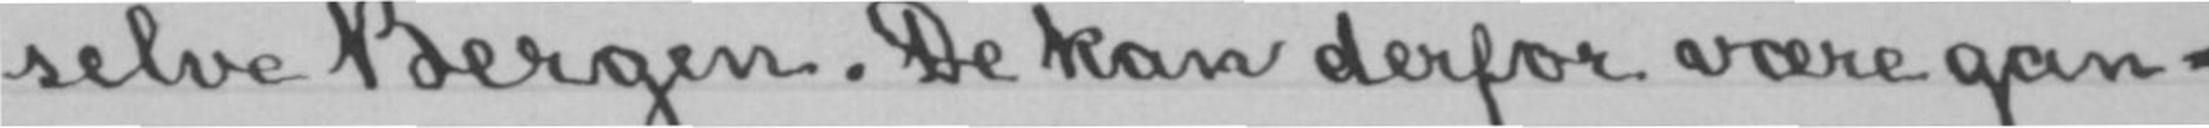selve Bergen. De kan derfor være gan-
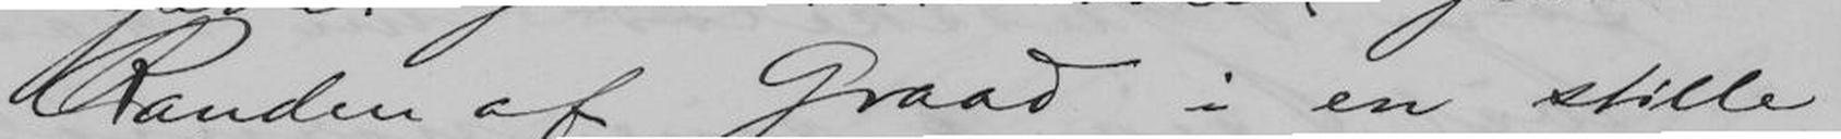Randen af Graad i en stille
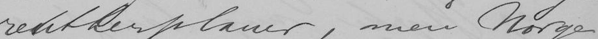reutherplaner, men Norge
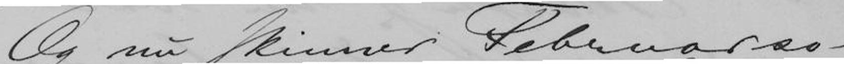Og nu skinner Februarso-
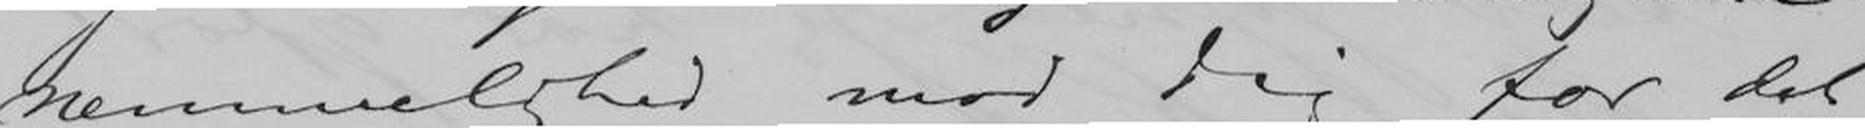nemmelighed mod dig for det
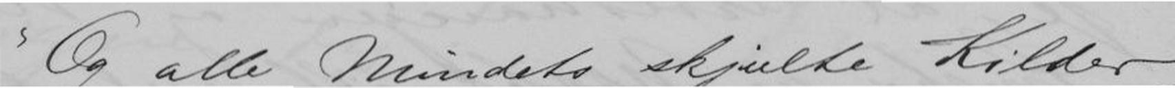"Og alle Mindets skjulte Kilder
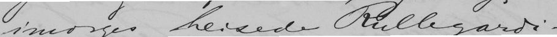imorges heisede Rullegardi-
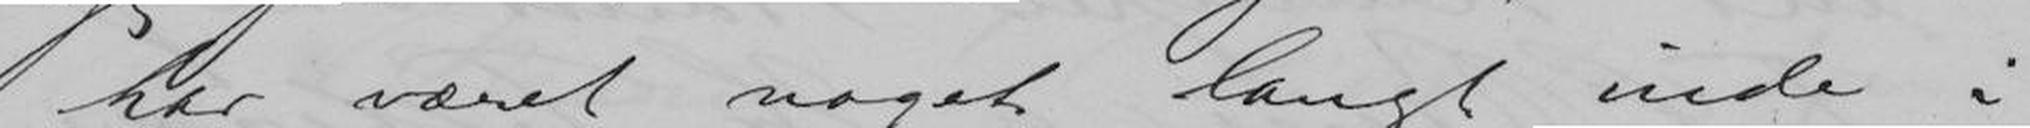har været noget langt inde i
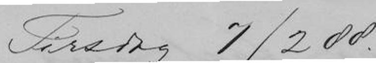Tirsdag 7/2 88.
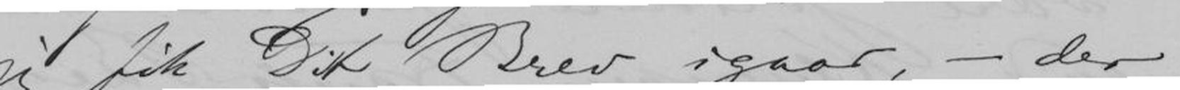jeg fik Dit Brev igaar, - der
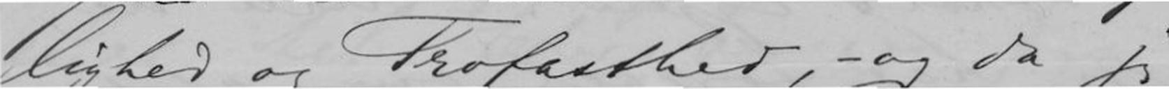lighed og Trofasthed, -og da jeg
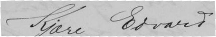Kjære Edvard!
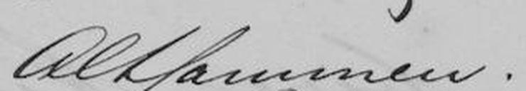Altsammen.
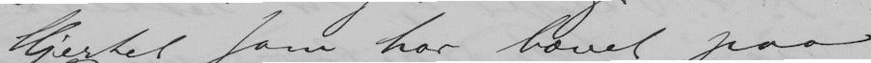Hjertet som har bævet paa
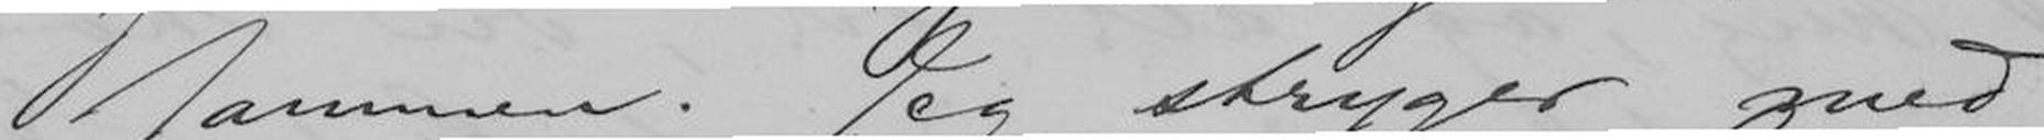sammen. Jeg stryger med
